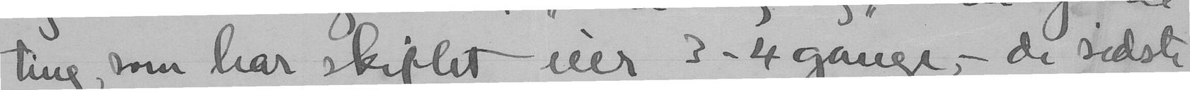ting, som har skiftet eier 3-4 gange, - de sidste
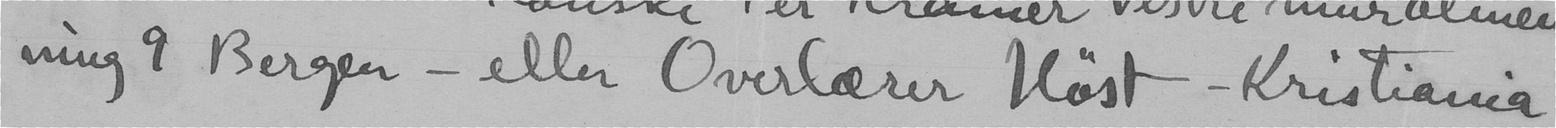ning 9 Bergen - eller Overlærer Høst - Kristiania
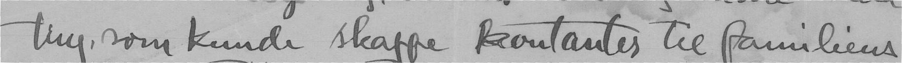ting, som kunde skaffe kontanter til familiens
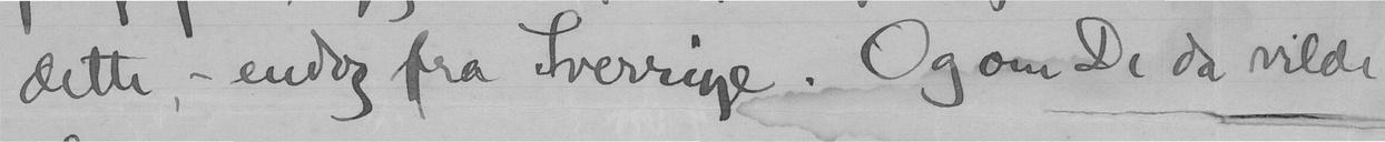dette, - endog fra Sverrige. Og om De da vilde
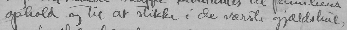ophold og til at stikke i de værste gjældshul,
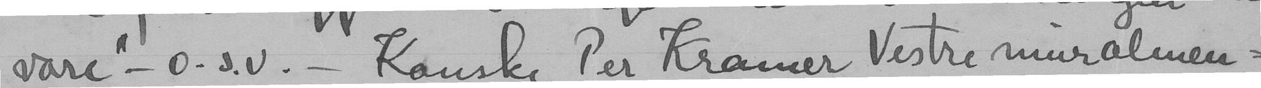vare" - o.s.v. - Kanske Per Kramer Vestre muralmen-
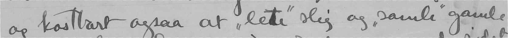og kostbart ogsaa at "lete" slig og "samle" gamle
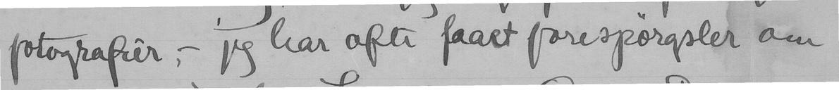fotografier, - jeg har ofte faaet forespørgsler om
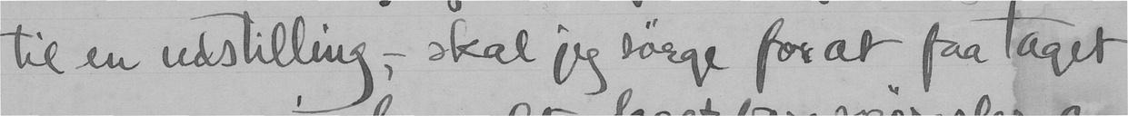til en udstilling, - skal jeg sørge for at faa taget
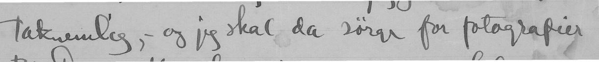taknemlig, - og jeg skal da sørge for fotografier
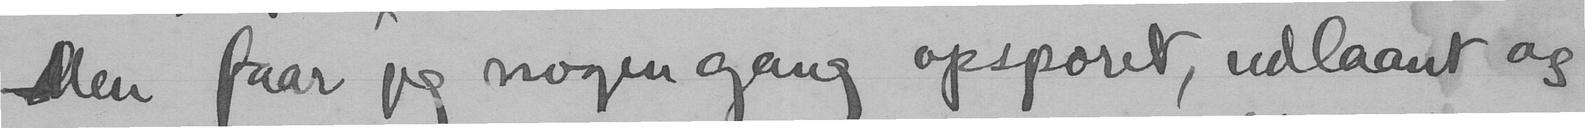Men faar jeg nogen gang opsporet, udlaant og
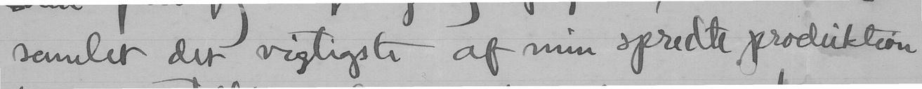samlet det vigtigste af min spredte produktion
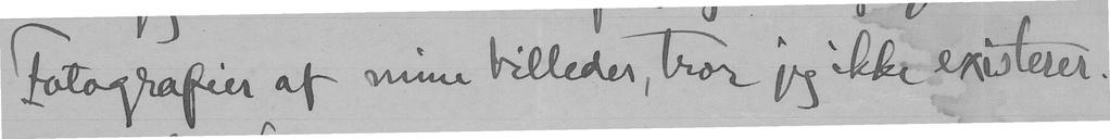Fotografier af mine billeder, tror jeg ikke existerer.
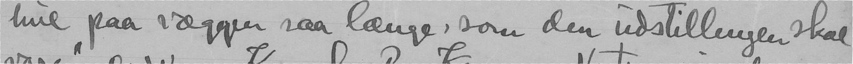hul paa væggen saa længe, som den udstillingen skal
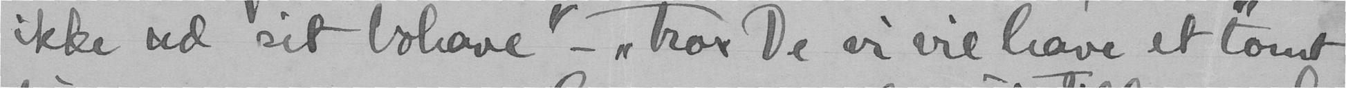ikke ud sit bohave" - "Tror De vi vil have et tomt
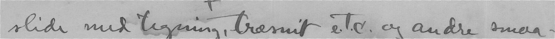slide med tegning, træsnit e.t.c. og andre smaa-
